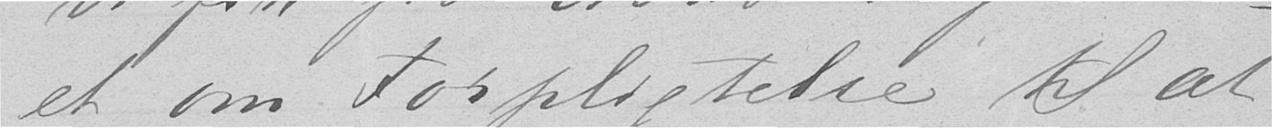et om Forpligtelse til at
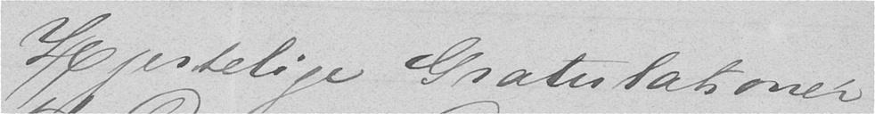Hjertelige Gratulationer
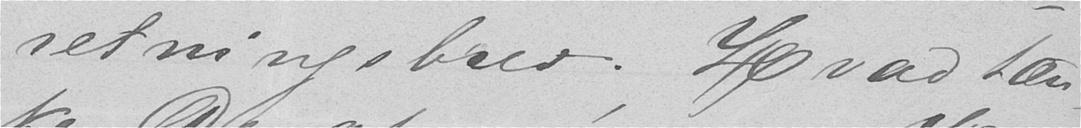retningsbrev. Hvad tæn-
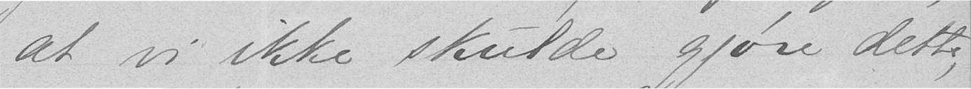at vi ikke skulde gjøre dette,
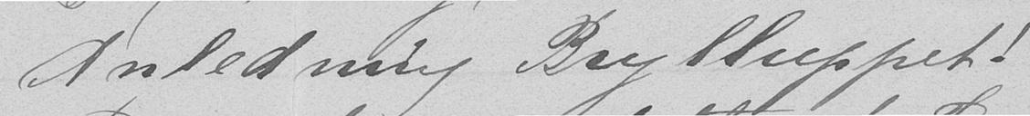Anledning Brylluppet!
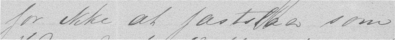for ikke at fastslaa, som
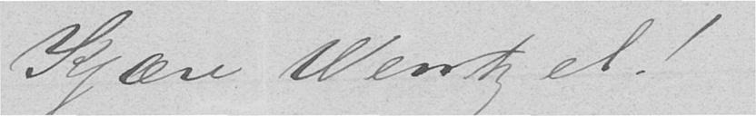Kjære Wentzel!
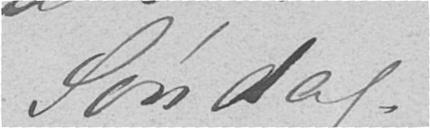Søndag
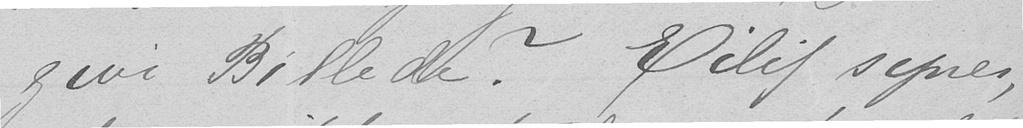give Billede? Eilif synes,
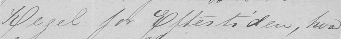Regel for Eftertiden, hvad
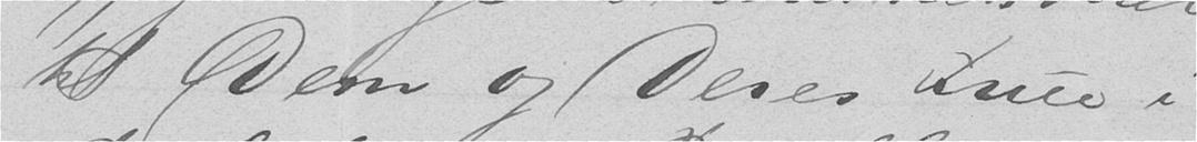til Dem og Deres Frue i
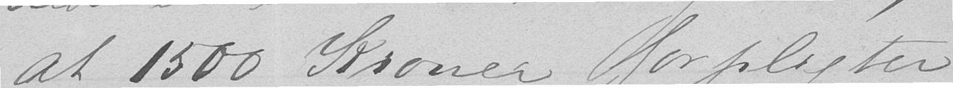at 1500 Kroner forpligter
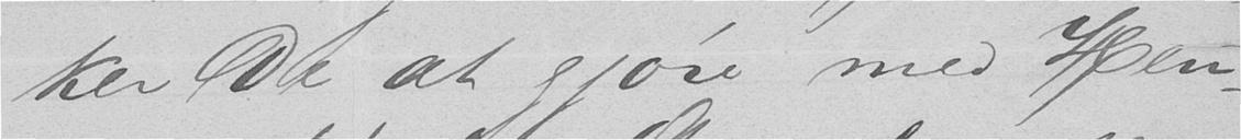ker de at gjøre med Hen-
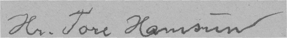Hr. Tore Hamsun
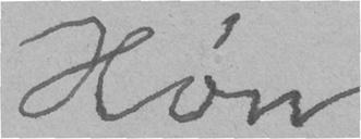Høn
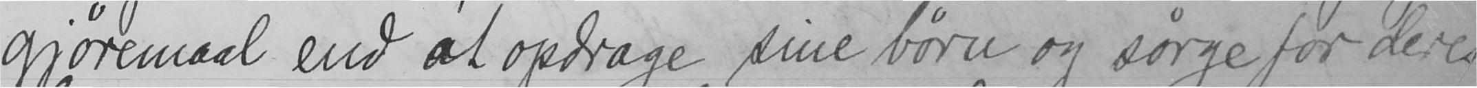gjøremaal end at opdrage sine børn og sørge for deres
